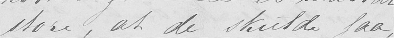store, at de skulde faa
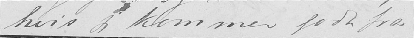hvis jeg kommer godt fra
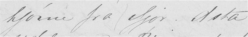hjørne fra ifjor. Asta
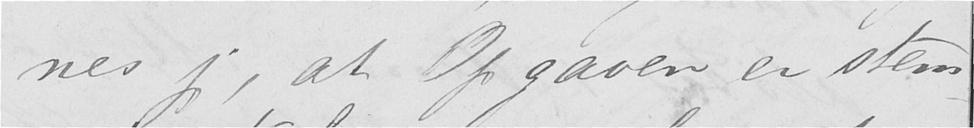nes jeg, at Opgaven er stem-
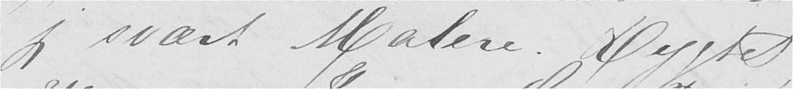jeg svært Malere. Rygtet
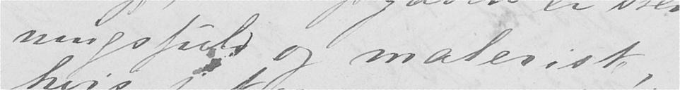ningsfund og malerisk,
